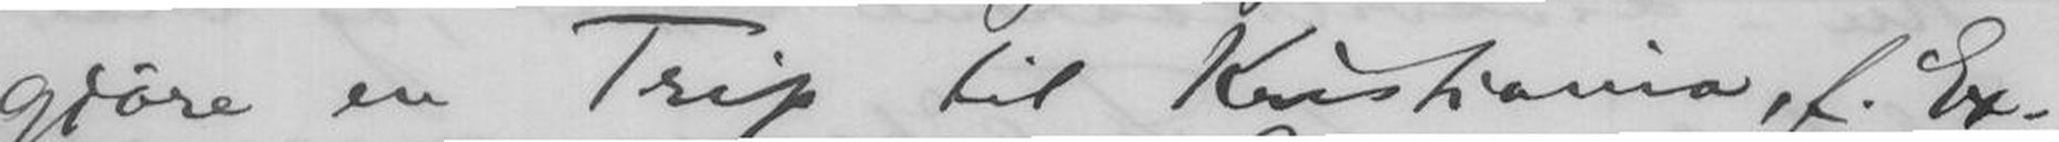gjøre en Trip til Kristiania, f. Ex.
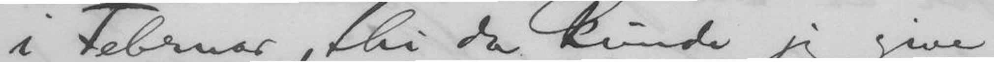i Februar, thi da kunde jeg give
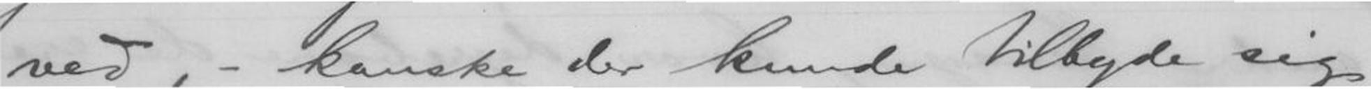ved, - kanske der kunde tilbyde sig
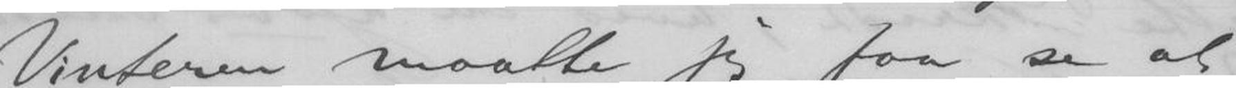Vinteren maatte jeg faa se at
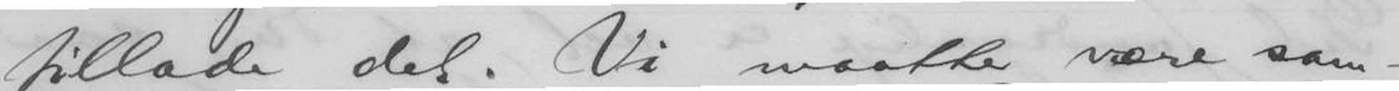tillade det. Vi maatte være sam-
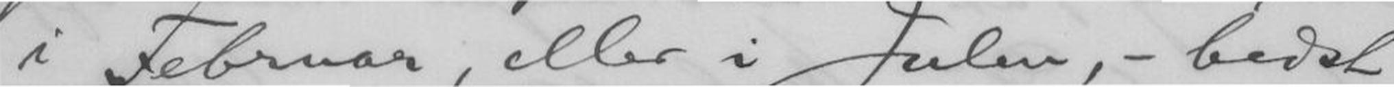i Februar, eller i Julen, - bedst
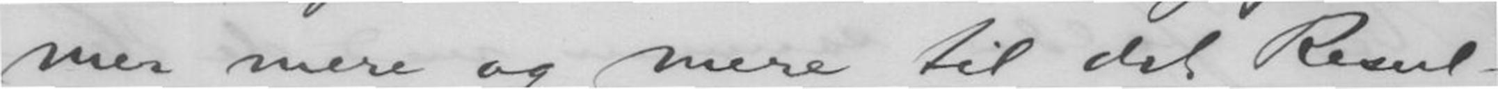mer mere og mere til det Resul-
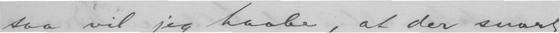saa vil jeg haabe, at der snart
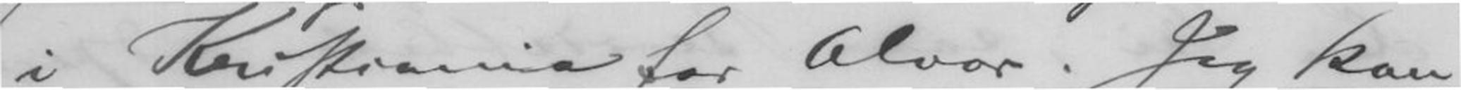i Kristiania for Alvor. Jeg kan
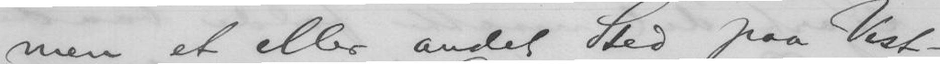men et eller andet Sted paa Vest-
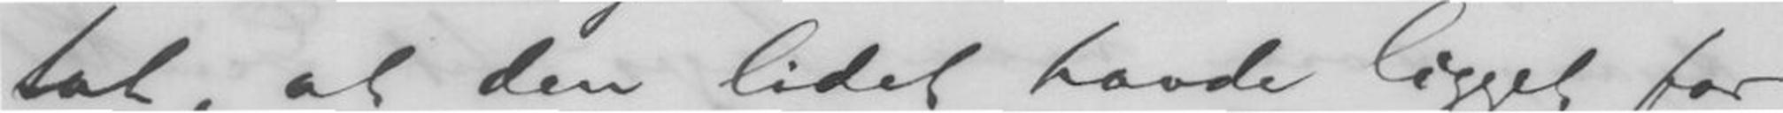tat, at den lidet havde ligget for
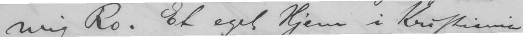mig Ro. Et eget Hjem i Kristiania
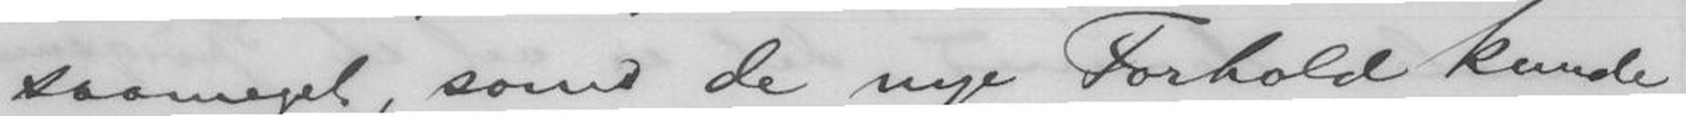saameget, som de nye Forhold kunde
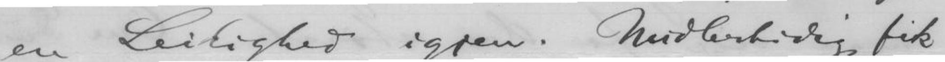en Leilighed igjen. Midlertidig fik
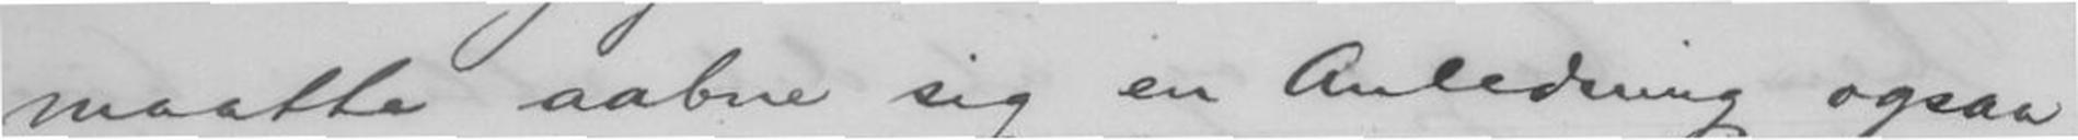maatte aabne sig en Anledning ogsaa
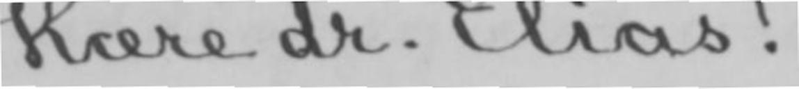Kære dr. Elias!
